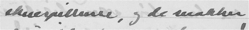skuespillerne, og de snakker
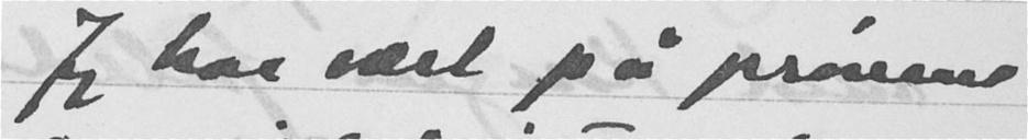Jeg har vært på prøvene
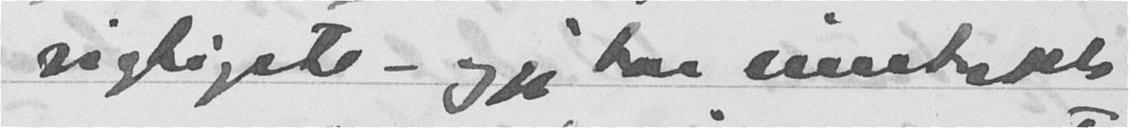vigtigste - og jeg har inntrykk
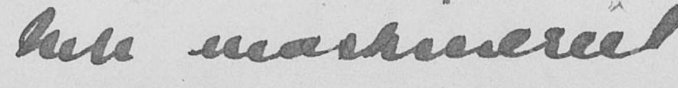hele maskineriet. -
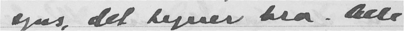egus, det begeier bro. Alle
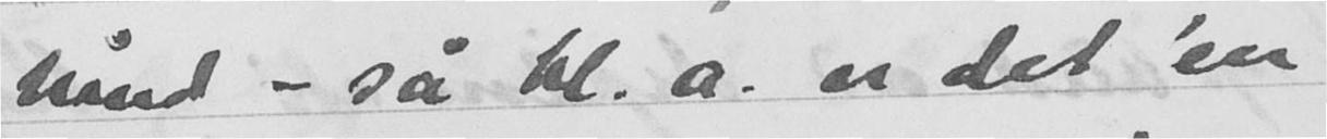hånd - så bl. a. er det en
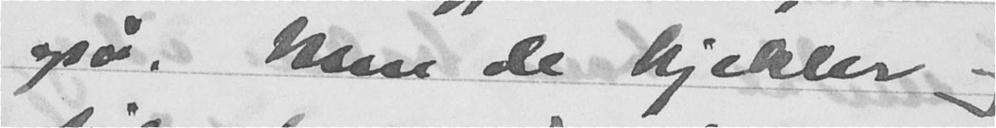også. Men de kjekler og
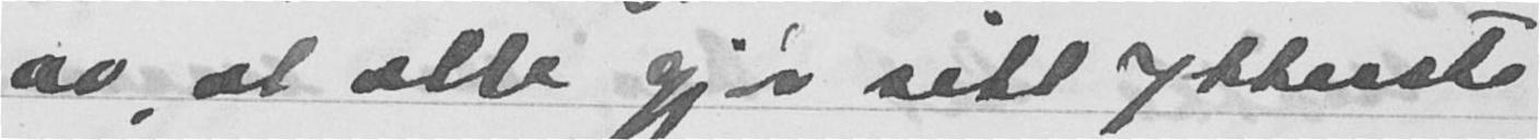av at alle gjør sitt ytterste
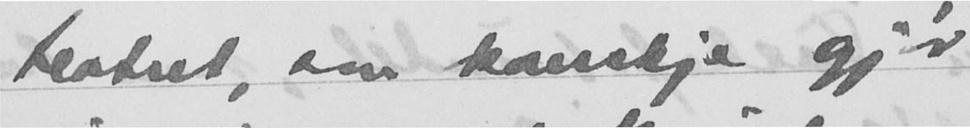teatret, som kanskje gjør
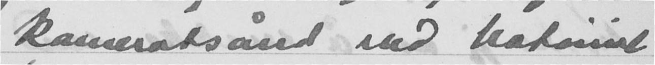kameratsånd end katoniet
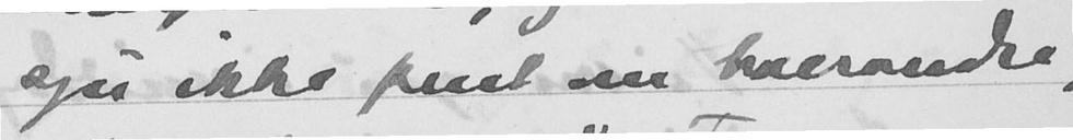også ikke pent om hverandre,
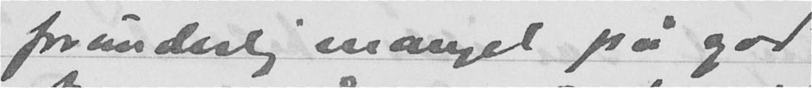forunderlig mangel på god
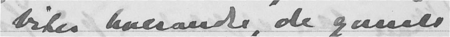biter hverandre, de gamle
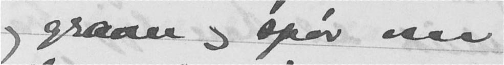og graver og spør om
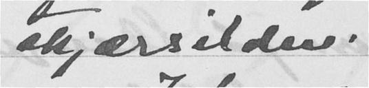skjærsilden.
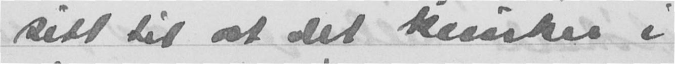sitt til at det knirker i
